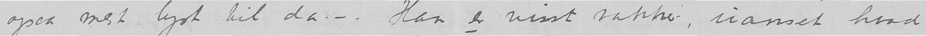ogsaa mest lyst til da. –. Han er visst vakker, uanset hvad
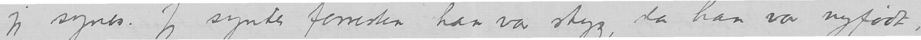jeg synes. Jeg syntes forresten han var stygg, da han var nyfødt,
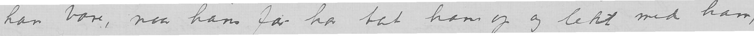han bare, naar hans far har tat ham op og lekt med ham,
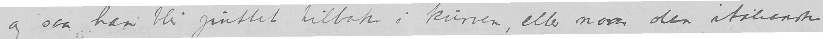og saa han blir puttet tilbake i kurven, eller naar den italienske
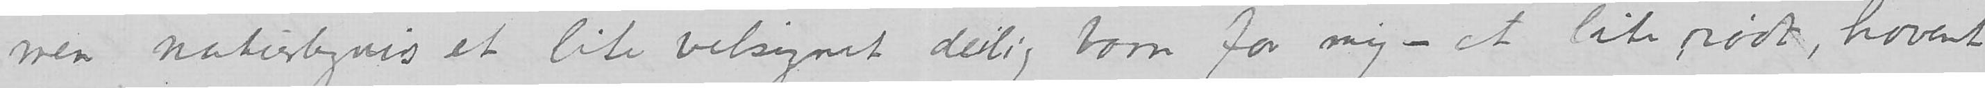men naturligvis et lite velsignet deilig barn for mig – et lite rødt, hovent
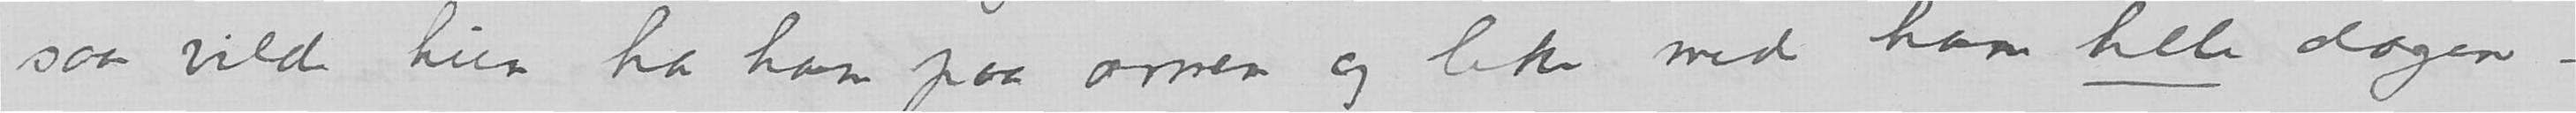saa vilde hun ha ham paa armen og leke med ham hele dagen –
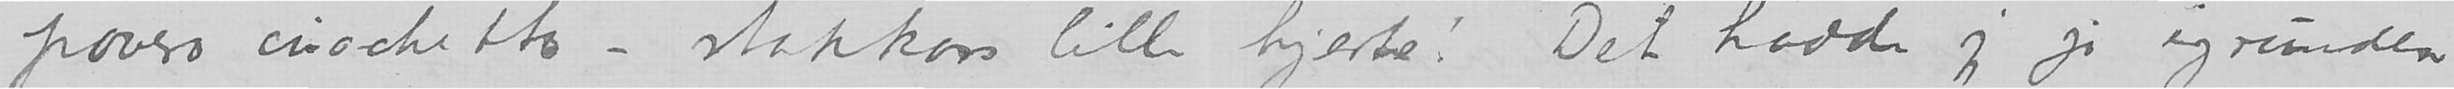povero ciochetto – stakkars lille hjerte! Det hadde jeg jo igrunden
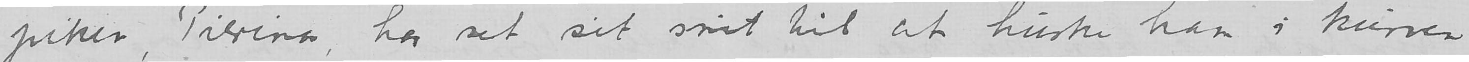piken, Pierina, har set sit snit til at huske ham i kurven
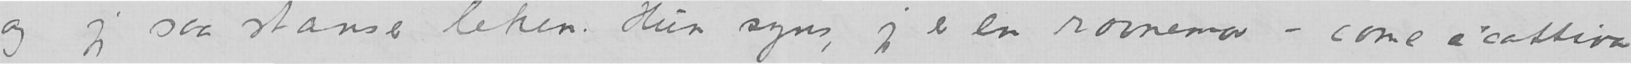og jeg saa stanser leken. Hun synes, jeg er en ravnemor – come e’cattiva
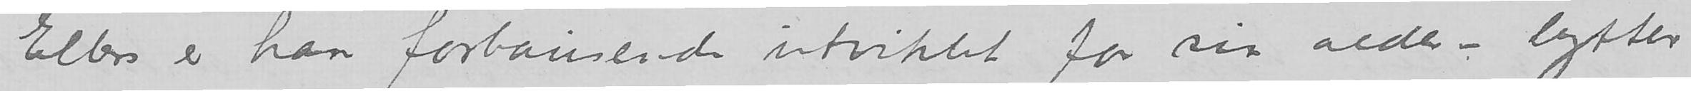Ellers er han forbausende utviklet for sin alder – lytter
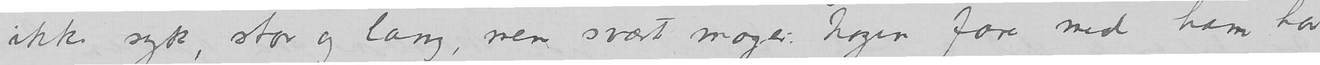ikke syk, stor og lang, men svært mager. Nogen fare med ham har
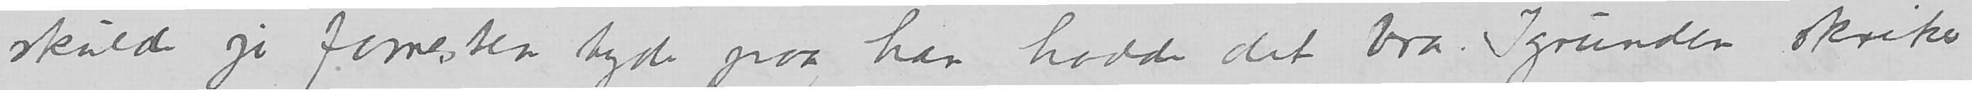skulde jo forresten tyde paa han hadde det bra. Igrunden skriker
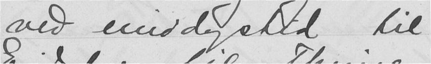ved middagstid til
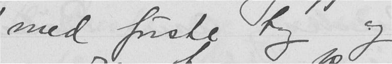med første tog og
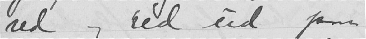red og red ud paa
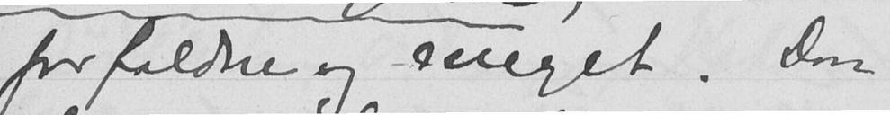forfaldne og meget. Don
